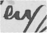en
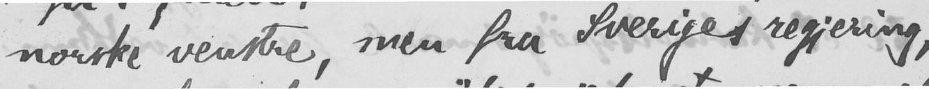norske venstre, men fra Sveriges regjering,
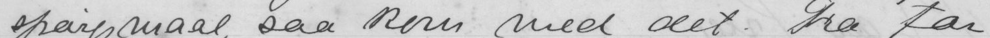spørgsmaal, saa kom med det. Fra Far
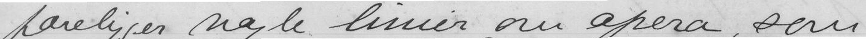foreligger nogle linier om opera, som
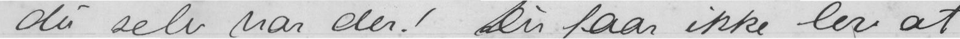du selv var der! Du faar ikke lov at
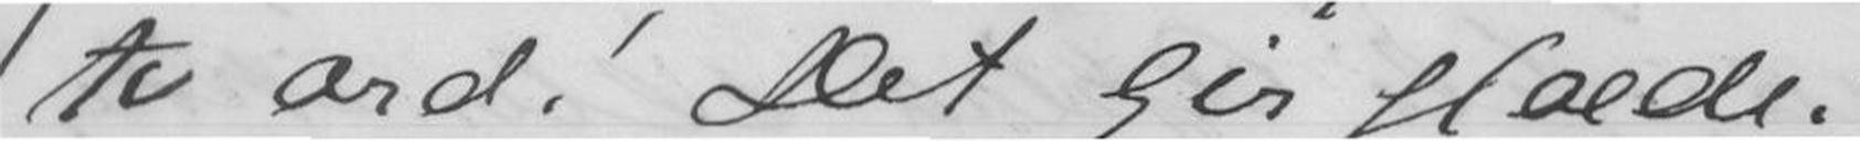to ord! Det gir glæde!
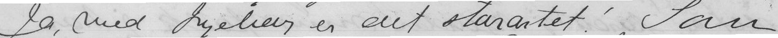Ja, med Ingeborg er det storartet! Som
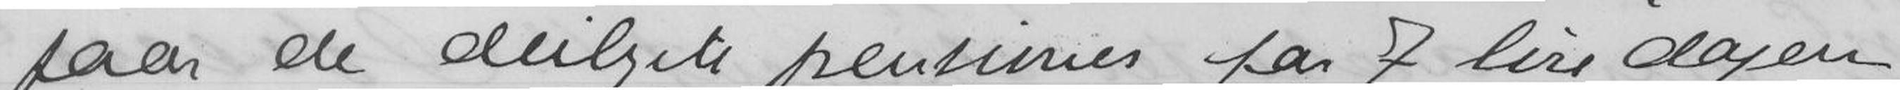faar de deiligste pensioner for 7 lire dagen
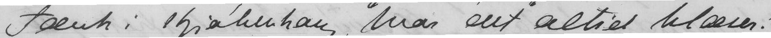Tænk i Kjøbenhavn, hvor det altid blæser!
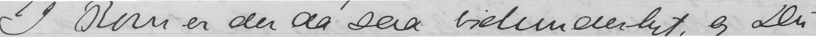I Rom er der saa vidunderligt, og Du
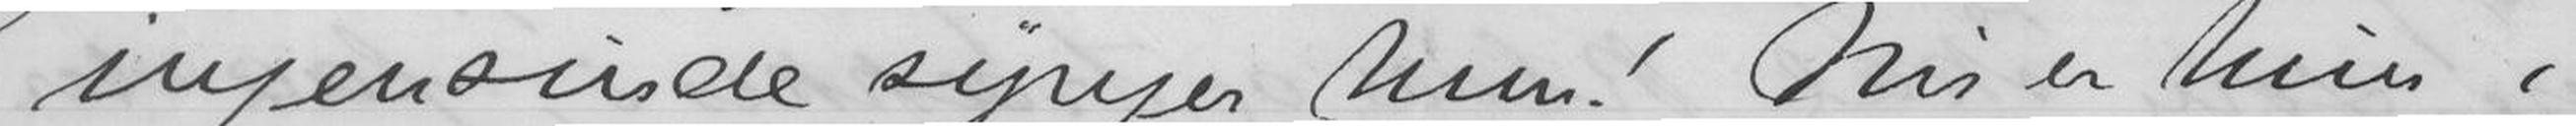ingensinde synger hun! Nu er hun i
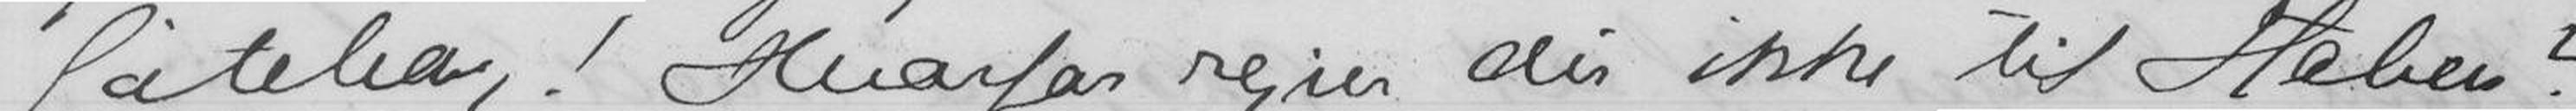Gøteborg! Hvorfor rejser du ikke til Italien?
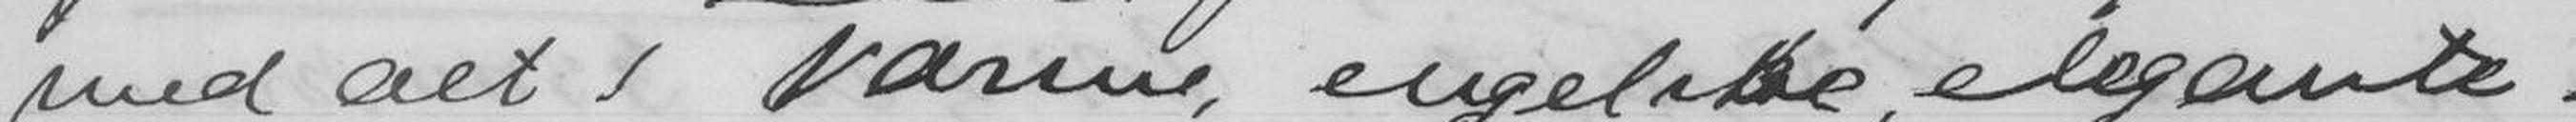med alt Varme, engelske, elegante!
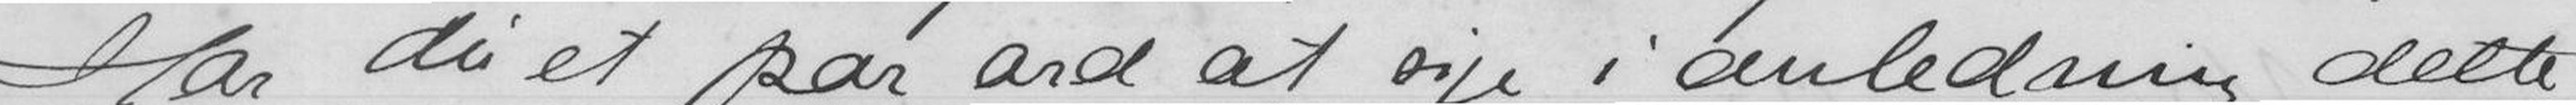Har du et par ord at sige i anledning dette
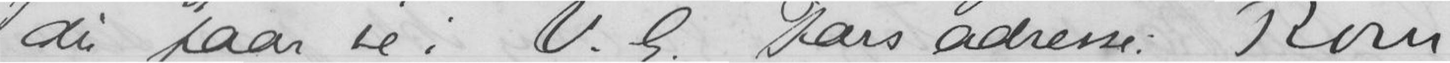du faar se i V.G. Fars adresse: Rom
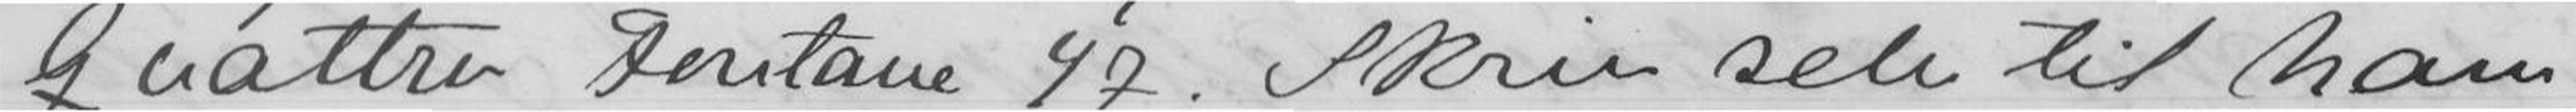Quattro Fontane 47. Skriv selv til ham
